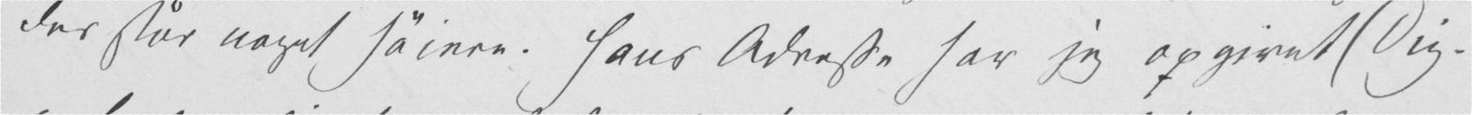der står noget høiere. Hans Adresse har jeg opgivet Dig.
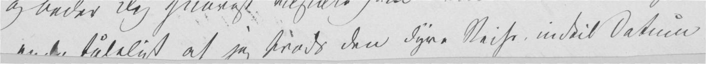endnu tåleligt at jeg trods den dyre Reise, indtil Datum
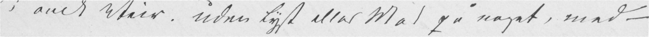i ondt Veir. uden Lyst eller Mod på noget, med -
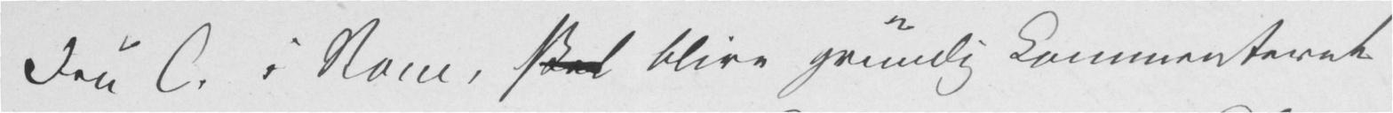Fru C. i Rom, skal blive grundig kommenteret
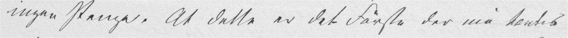ingen Penge. At dette er det Første der må tænkes
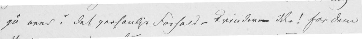gå over i det personlige Forhold – Kvinderne ikke! for dem
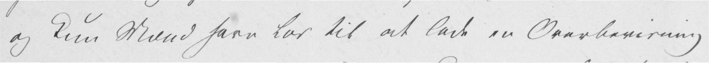og kun Mænd have Lov til at lade en Overbevisning
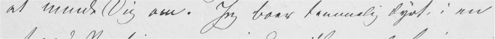at minde Dig om. Jeg boer temmelig dyrt, i en
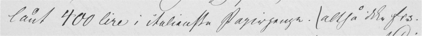lånt 400 Lire i italienske Papirpenge. (altså ikke frs.
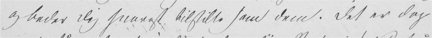og beder Dig snarest tilstille ham dem. Det er dog
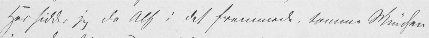Her sidder jeg da Alf i det fremmede tomme München
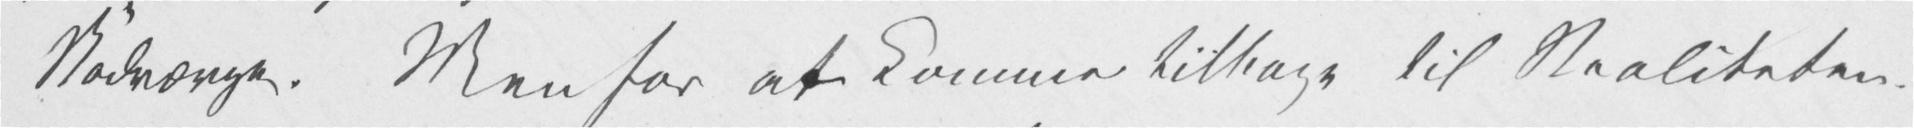Nødværge. Men for at komme tilbage til Realiteten
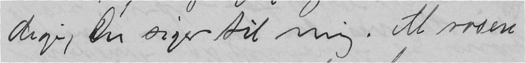dige, Du siger til mig. Al rosen
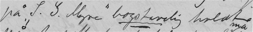på "S. G. Myre" bogstavelig holdt
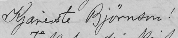Kjæreste Bjørnson!
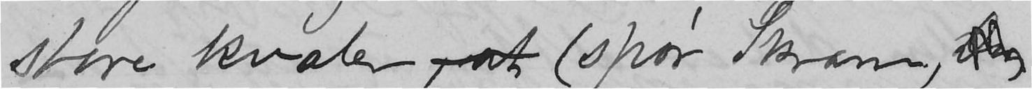store kvaler at (spør Skram, Dog
